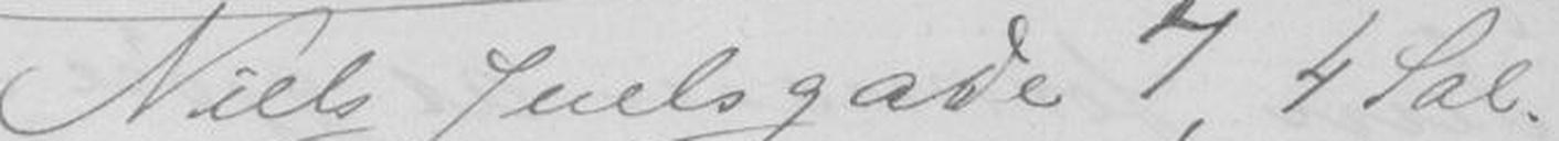Niels Juelsgade 7, 4 Sal.
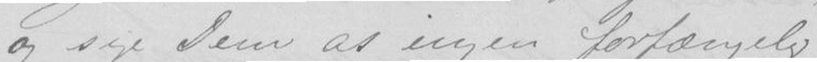og sige Dem at ingen forfængelige
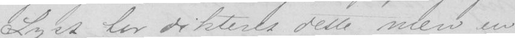Lyst har dikteret dette, mæn en
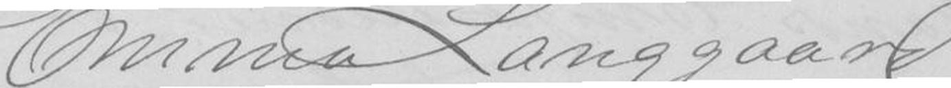Emma Sanggaard
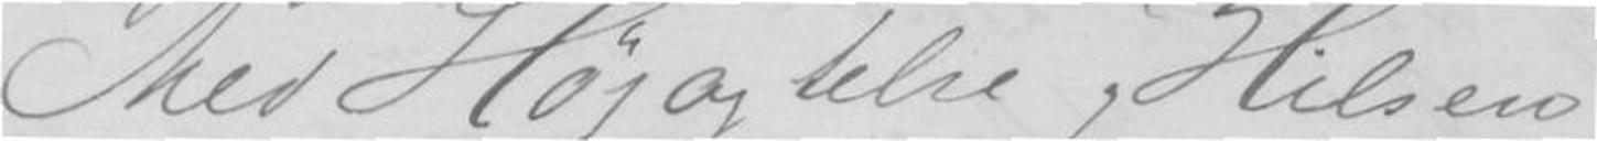Med Højagtelse og Hilsen
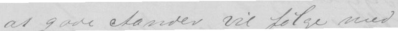at Aander vil følge med
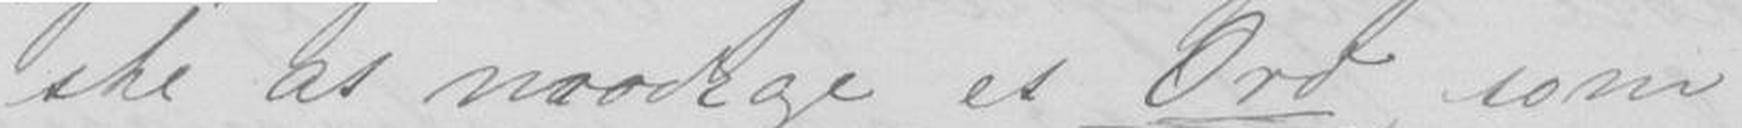ske at mo et Ord som
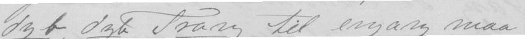dyb dyb Trang til engang maa-
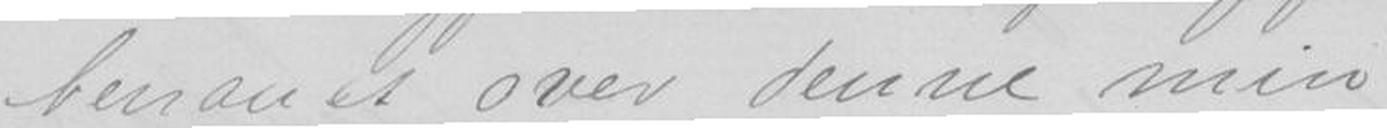over denne min
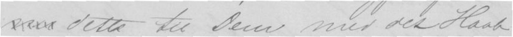dette til Dem med det Haab,
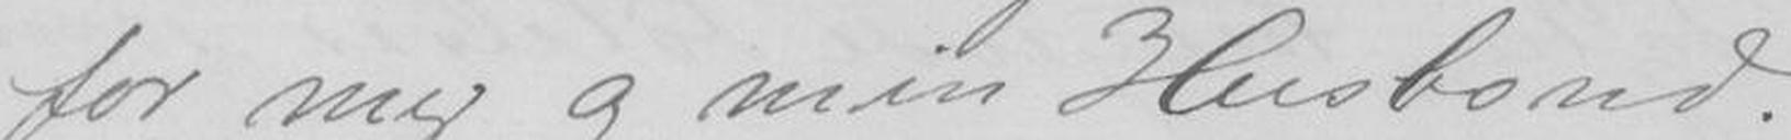for mig og min Husbond.
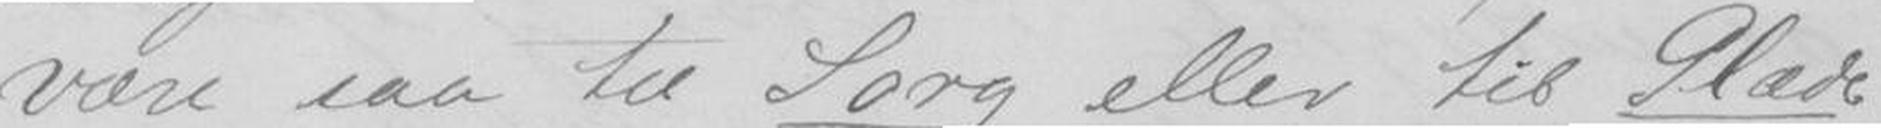være saa til Sorg eller til Glæde
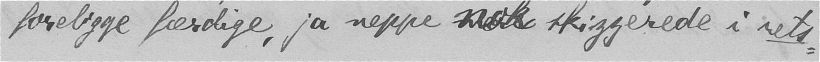foreligge færdige, ja neppe nok skizzerede i rets-
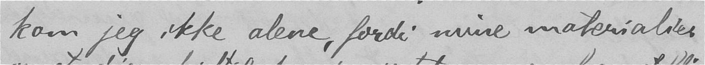kom jeg ikke alene, fordi mine materialier
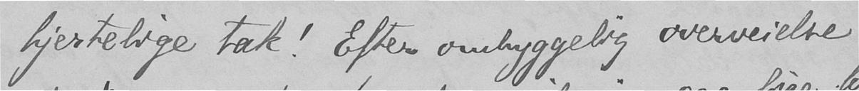hjertelige tak! Efter omhyggelig overveielse
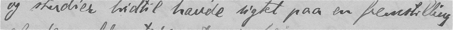og studier hidtil havde sigtet paa en fremstilling
